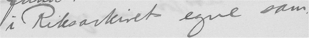i Riksarkivets egne sam-
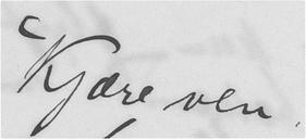Kjære ven!
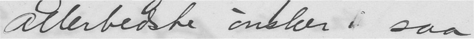allebedste ønsker i saa
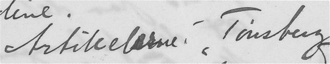Artikelerne i "Tønsberg
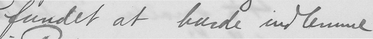fundet at burde indlemme
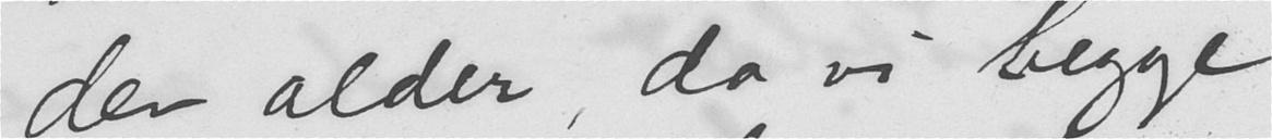den alder, da vi begge
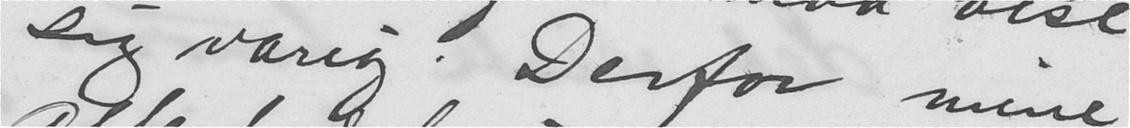sig varig. Derfor mine
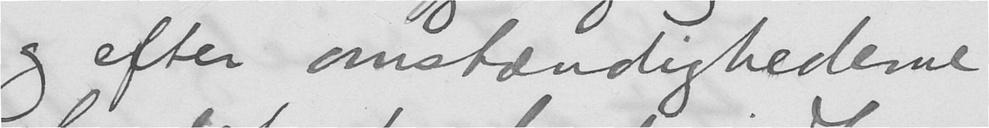og efter omstændighederne
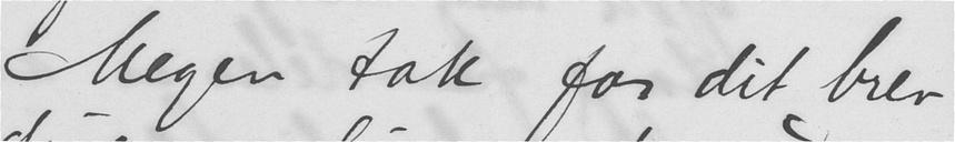Megen tak for dit brev
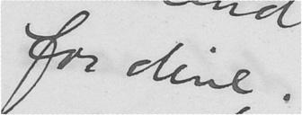for dine.
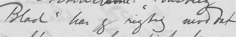Blad" har jeg rigtig modtat
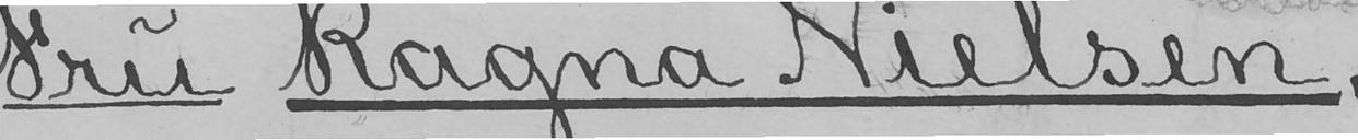Fru Ragna Nielsen,
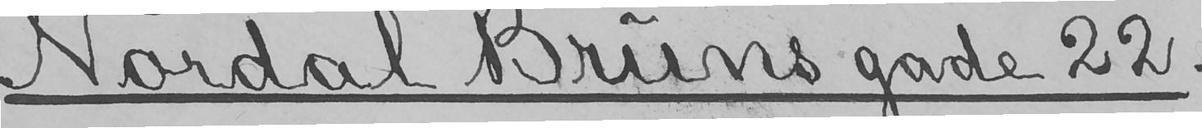Nordal Bruns gade 22.
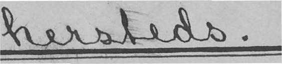hersteds.
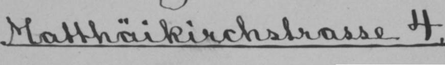Matthäikirchstrasse 4.
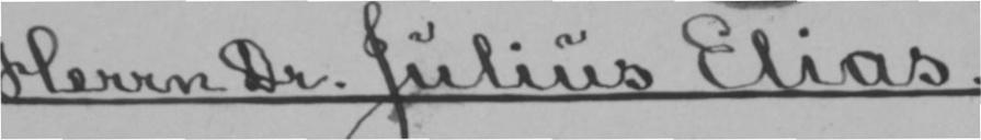Herrn Dr. Julius Elias.
